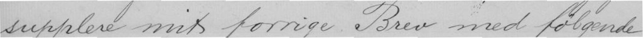supplere mit forrige Brev med følgende
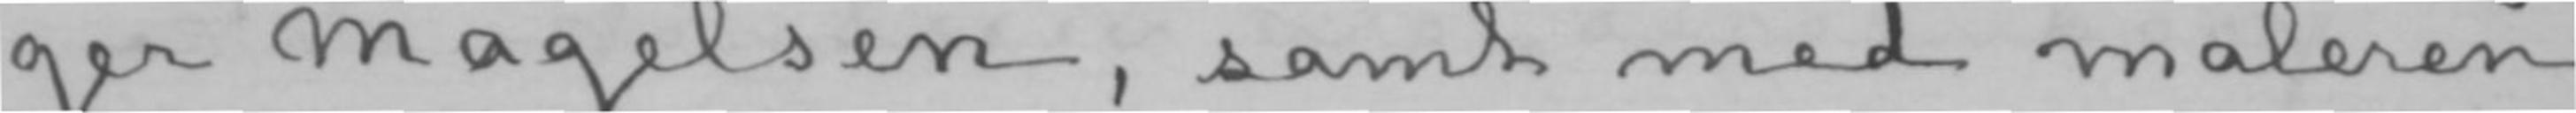ger Magelsen, samt med maleren
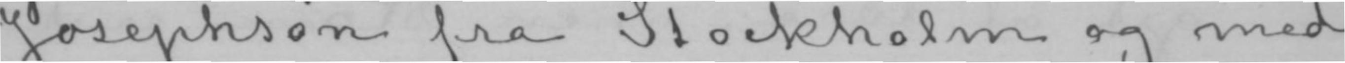Josephson fra Stockholm og med
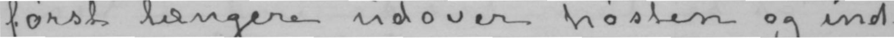først længere udover høsten og ind-
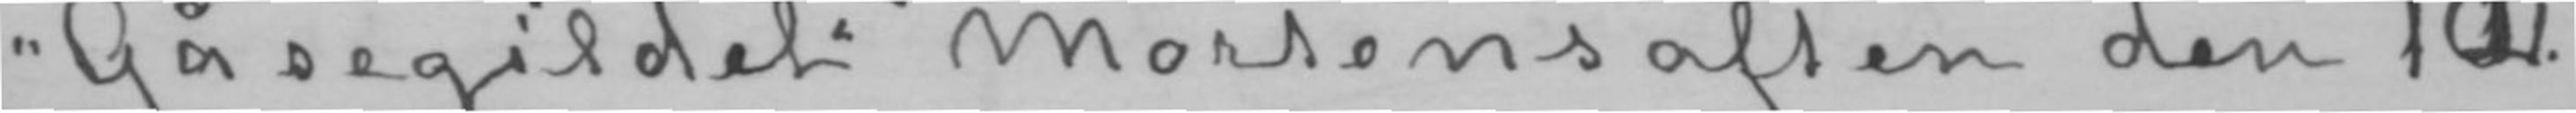«Gåsegildet» Mortensaften den 120.
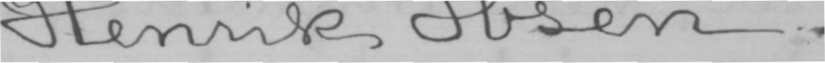Henrik Ibsen.
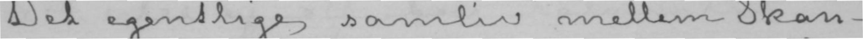Det egentlige samliv mellem Skan-
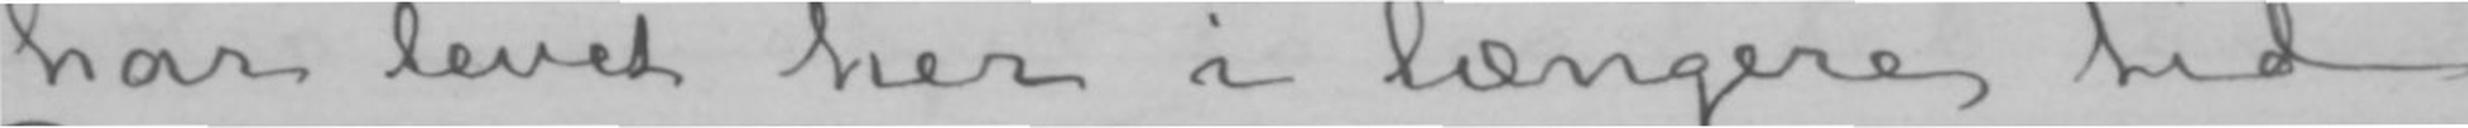har levet her i længere tid.
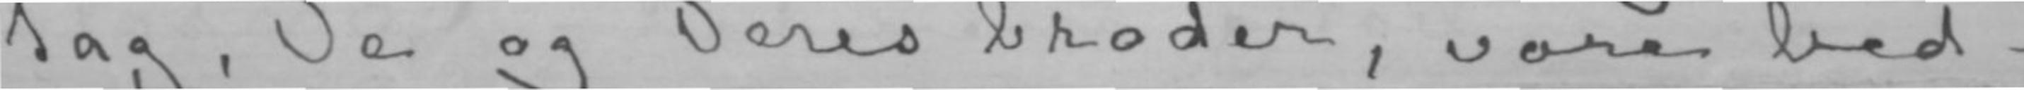tag, De og Deres broder, vore bed-
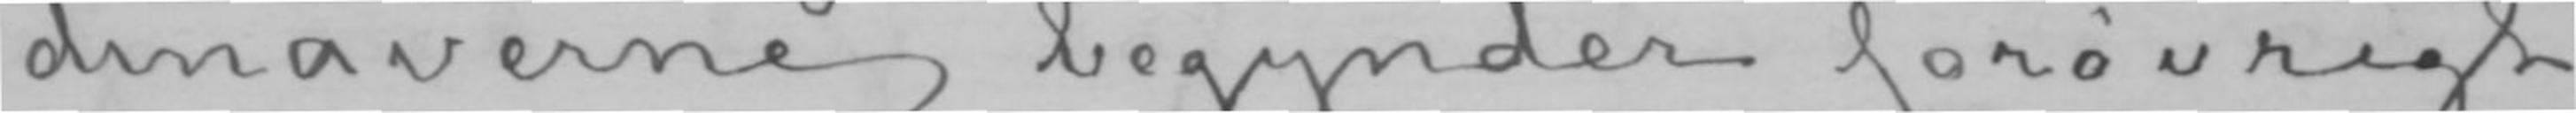dinaverne begynder forøvrigt
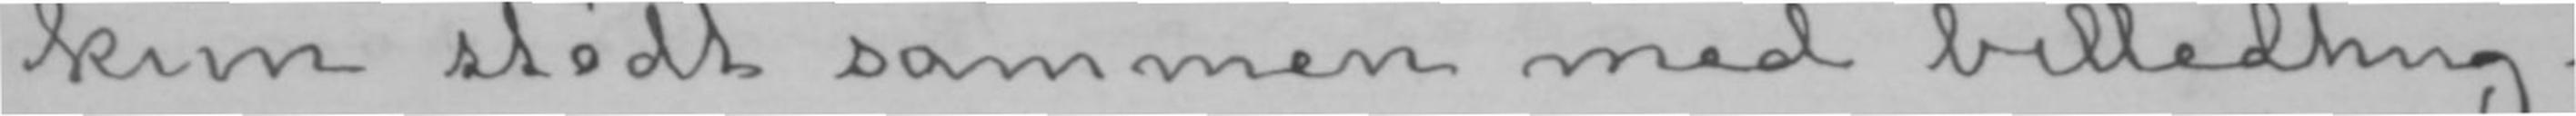kun stødt sammen med billedhug-
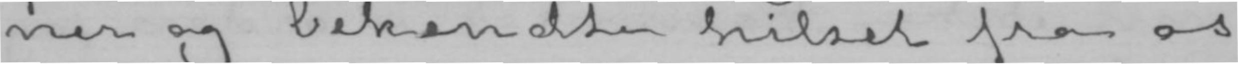ner og bekendte hilset fra os
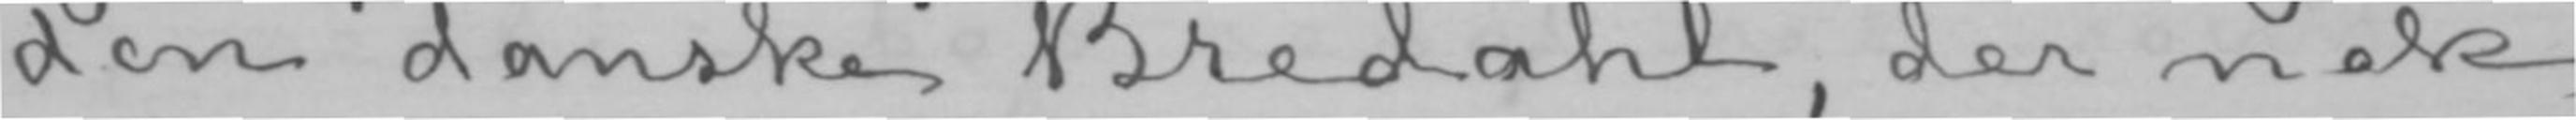den danske Bredahl, der nok
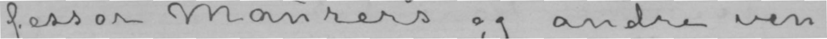fessor Maurers og andre ven-
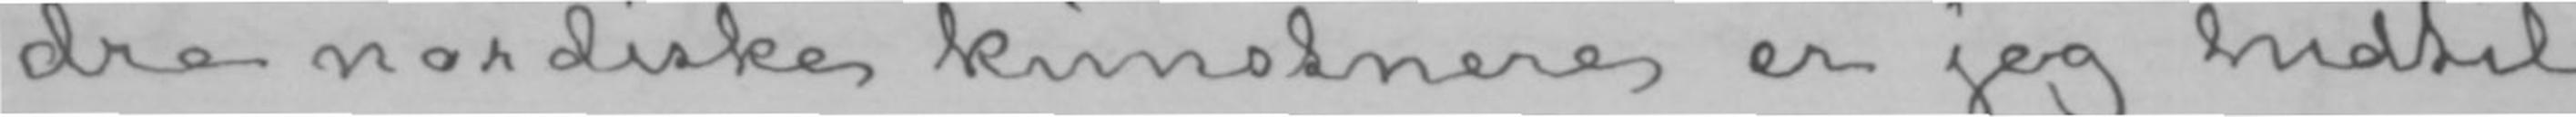dre nordiske kunstnere er jeg hidtil
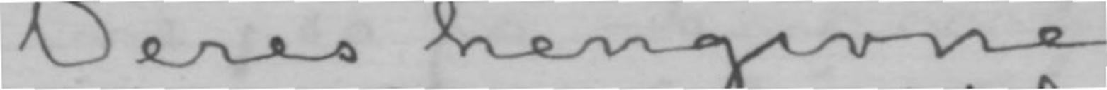Deres hengivne
